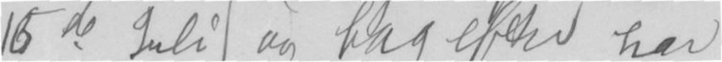15de Juli) og bagefter har
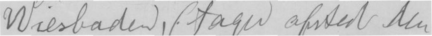Wiesbaden, (tager afsted den
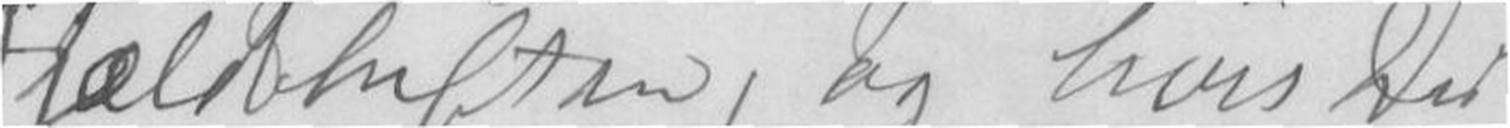Fjældluften, og hvis Du
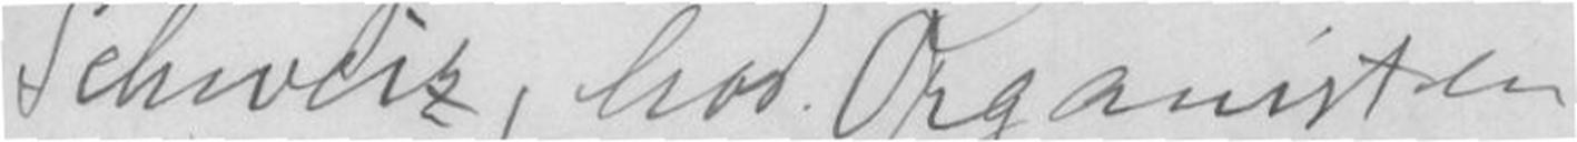Schweiz, hos Organisten
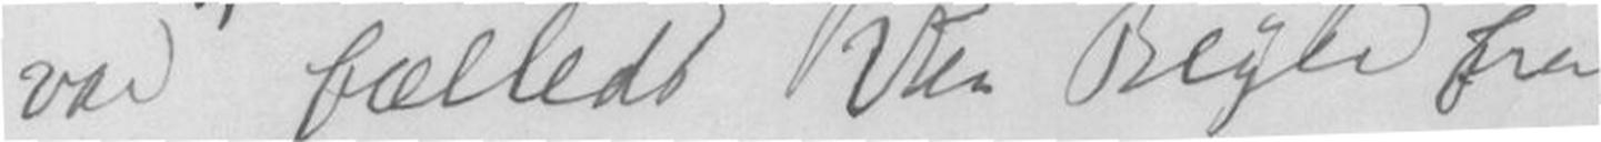vor fælleds Ven Beyer fra
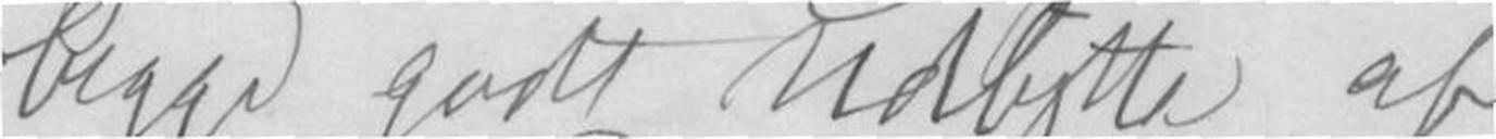begge godt Udbytte af
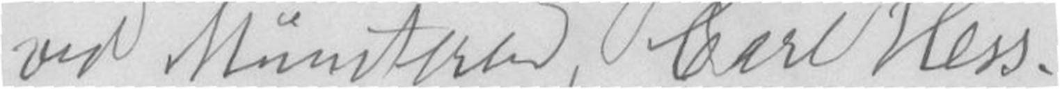ved Münstern, Carl Hess.
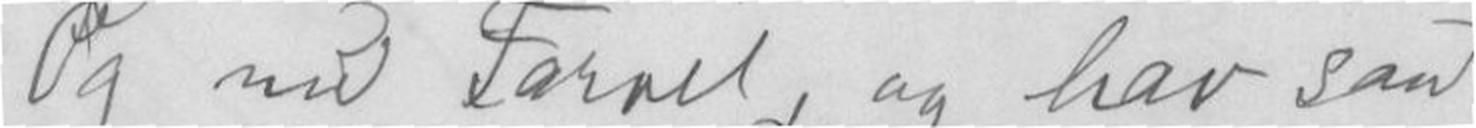Og nu Farvel, og hav saa
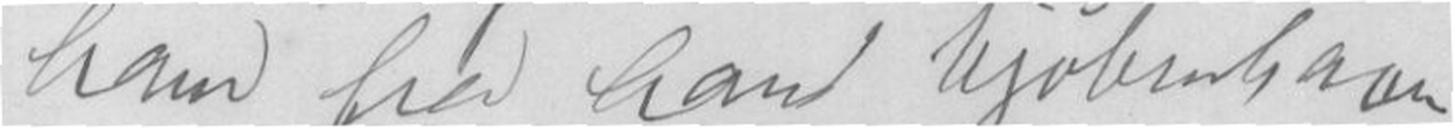ham fra hans kjøbenhavn-
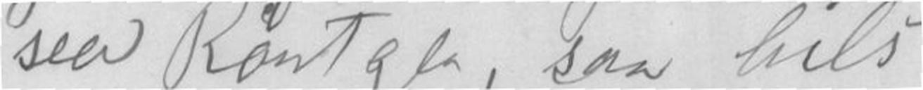seer Røntger, saa hils
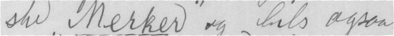ske Merker og hils ogsaa
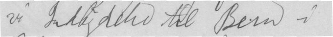vi Indbydelse til Bern i
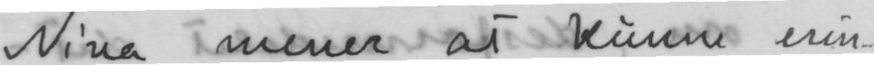Nina mener at kunne erin-
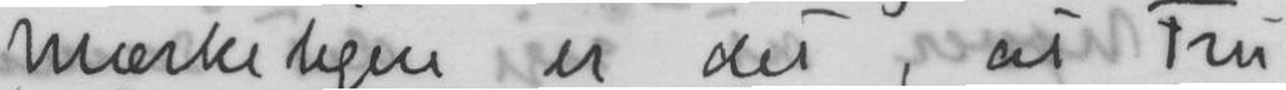Mærkeligen er det, at Fru
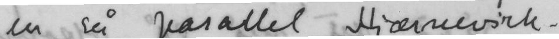en så parallel Hjærnevirk-
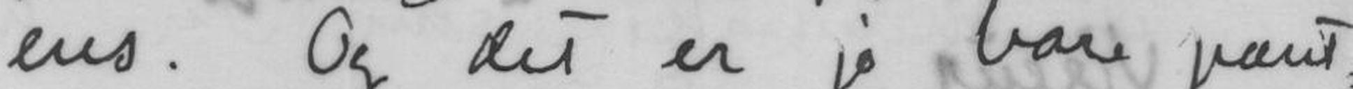ens. Og det er jo bare pænt
